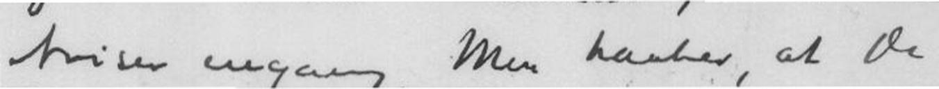Aviser engang. Men haaber, at De
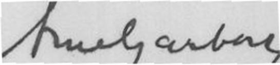Arne Garborg.
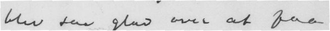blev saa glad over at faa
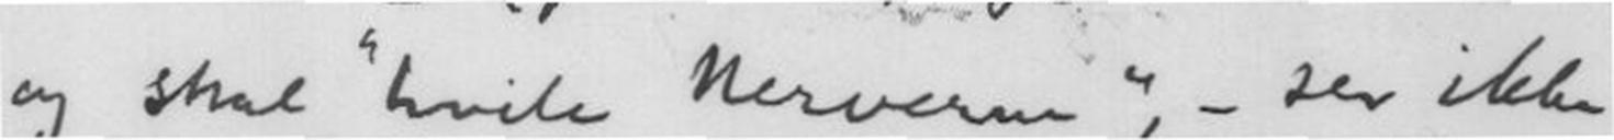og skal "hvile Nerverne", - ser ikke
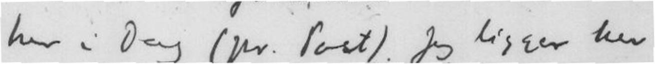her i Dag (pr. Post). Jeg ligger her
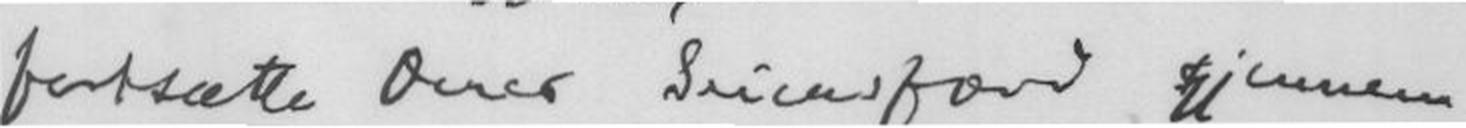fortsætte Deres Seiersfærd gjennem
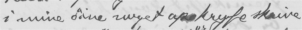i mine øine noget apokryfe skrive-
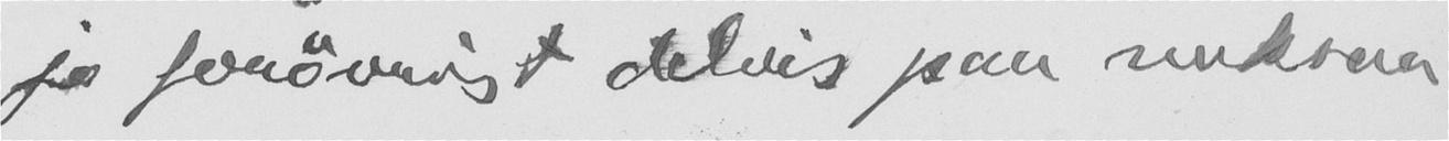jo forøvrigt delvis paa noksaa
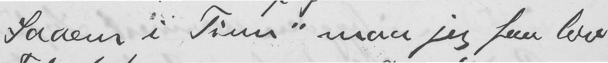Saaem i Tinn" maa jeg faa lov
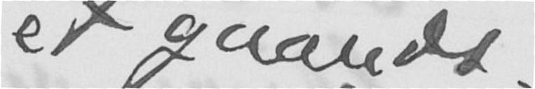et gaards-
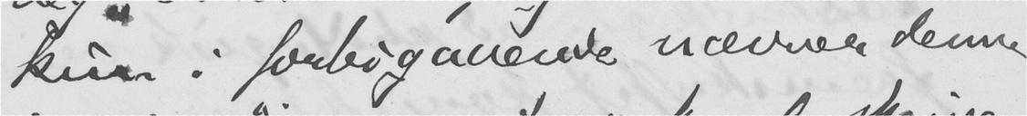kun i forbigaaende nævner denne
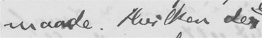maade. Hvilken der
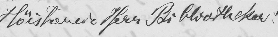Høistærede Herr Bibliothekar!
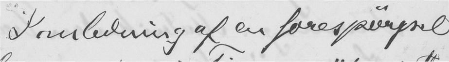I anledning af en forespørgsel
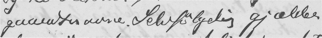gaardsnavne. Selvfølgelig gjælder
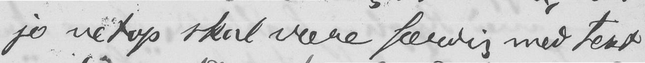jo netop skal være færdig med text
